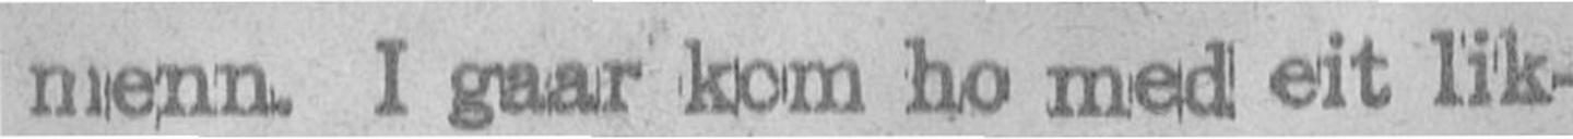nenn. I gaar kom ho med eit lik-
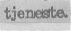tjeneste.
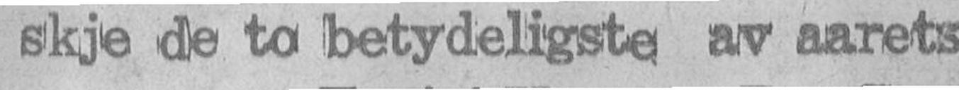skje de to betydeligste av aarets
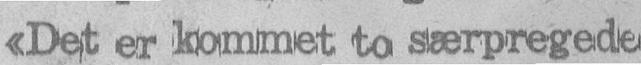«Det er kommet to særpregede
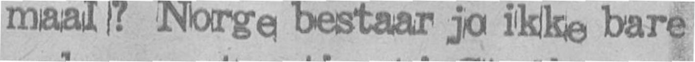maal? Norge bestaar jo ikke bare
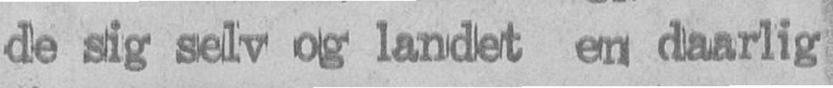de sig selv og landet en daarlig
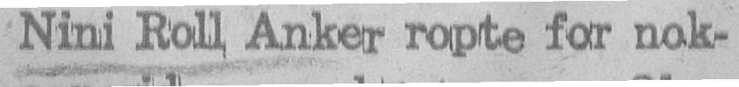Nini Roll Anker ropte for nok-
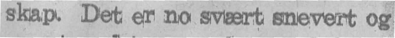skap. Det er no svært snevert og
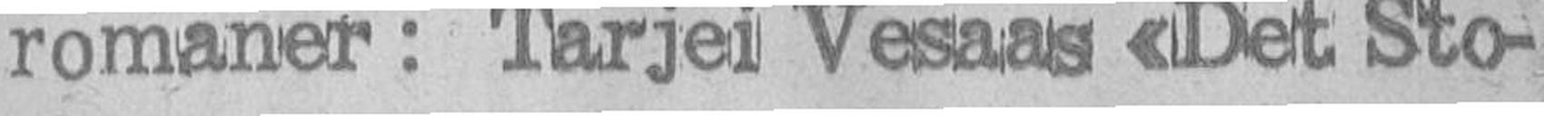romaner: Tarjei Vesaas «Det Sto-
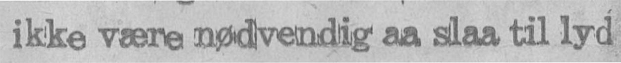ikke være nødvendig aa slaa til lyd
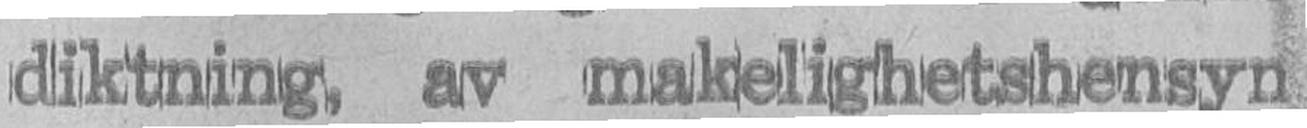diktning, av makelighetshensyn
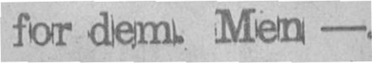for dem. Men —
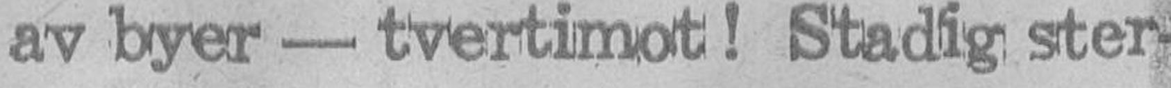av byer — tvertimot! Stadig ster-
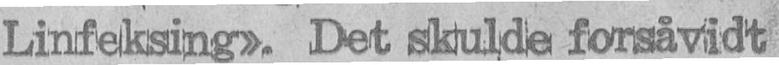Linfeksing». Det skulde forsåvidt
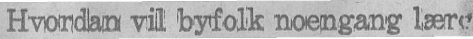Hvordan vil byfolk noengang lære
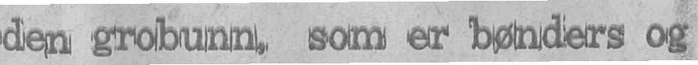den grobunn, som er bønders og
